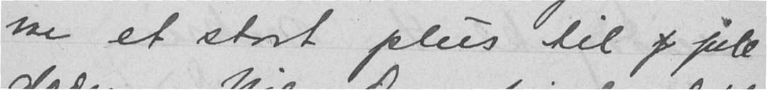var et stort plus til j jule
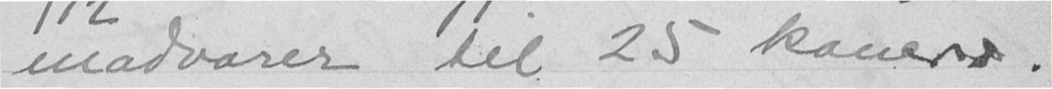madvarer til 25 koner d.
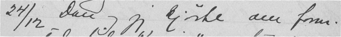24/12 Don og jeg kjørte om form.
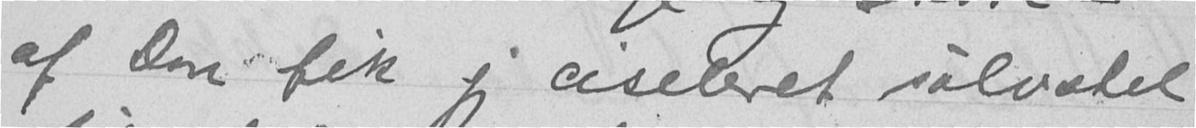af Don fik jeg ciseleret sølvstel
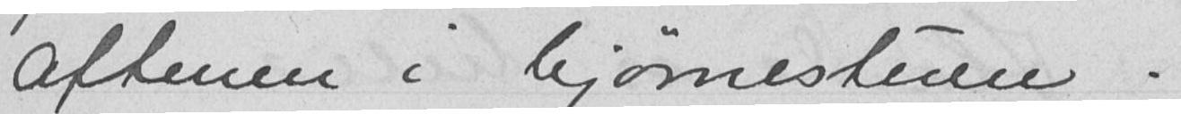aftenen i bjørnestuen.
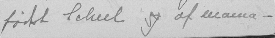født Scheel og af mama -
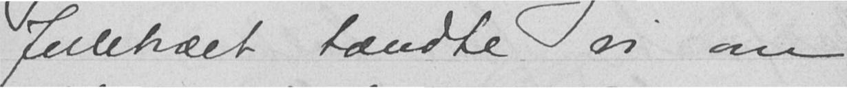Juletræet tændte vi om
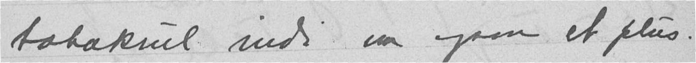tobakrul indi var ogsaa et plus.
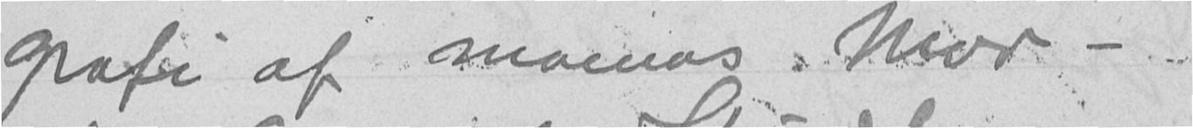grafi af mamas mor -
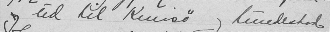og ud til Knivsø og lundestad
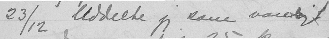23/12 Uddelte jeg som vanligt
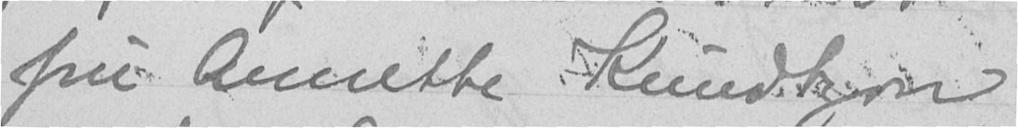fru Annette Knudtzon
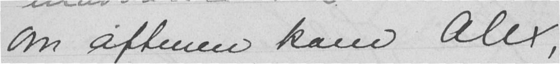Om aftenen kom Alex,
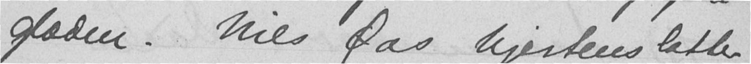glæden. Nils Øas hjertens latter
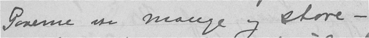Gaverne ere mange og store -
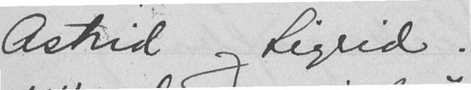Astrid og Sigrid.
